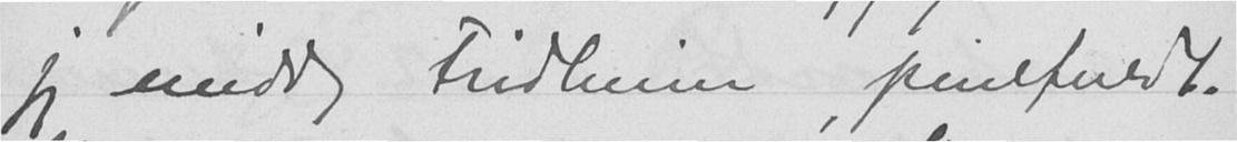jeg middag Fridheim, pinefuldt.
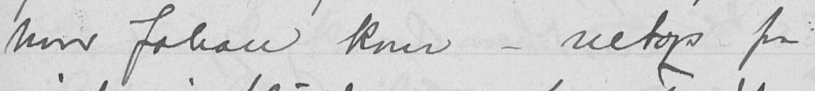hvor Johan kom - netop fra
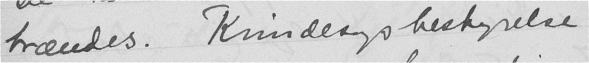brændes. Kvindesagsbestyrelse
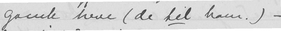gamle breve (de til ham.) -
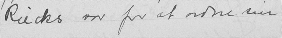Riecks var for at ordne sin
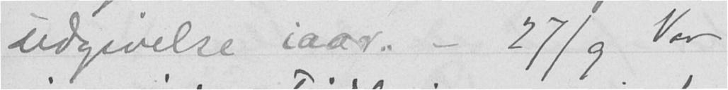udgivelse iaar. - 27/9 Var
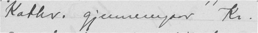Kathr. gjennemgaar Kr.
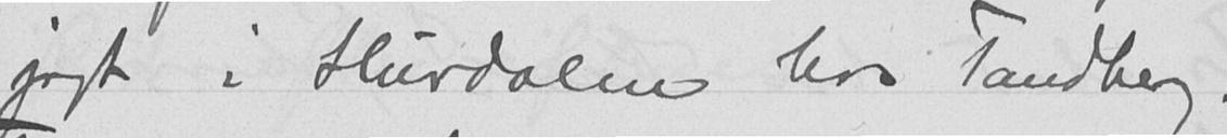jagt i Hurdalen hos Tandberg.
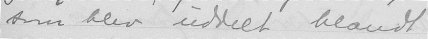som blev uddelt blandt
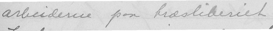arbeiderne paa træsliberiet
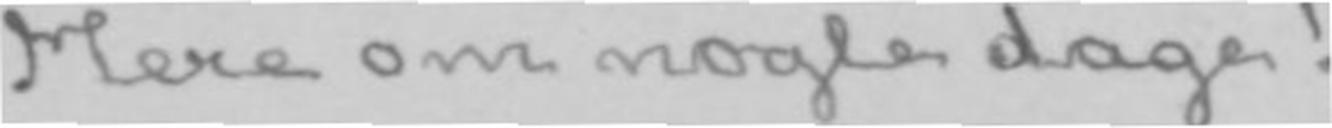Mere om nogle dage!
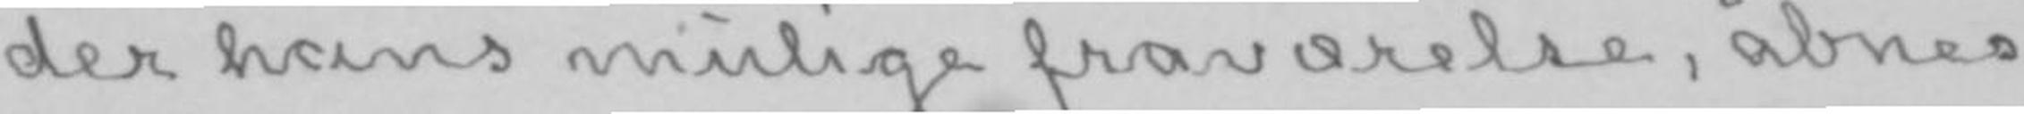der hans mulige fraværelse, åbnes
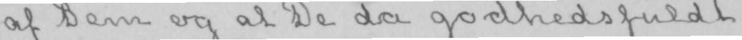af Dem og at De da godhedsfuldt
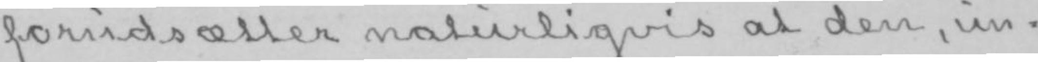forudsætter naturligvis at den, un-
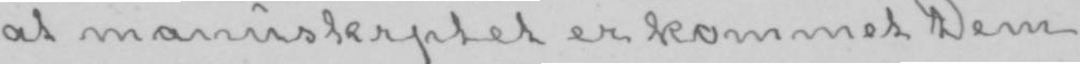at manuskrptet er kommet Dem
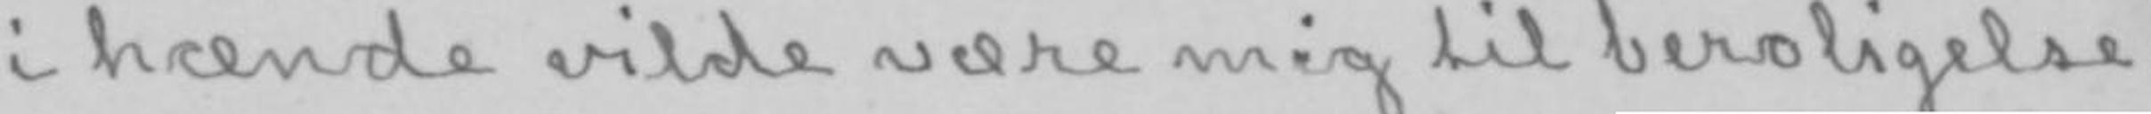i hænde vilde være mig til beroligelse.
